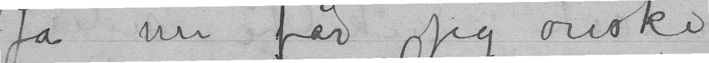Ja nu får jeg ønske
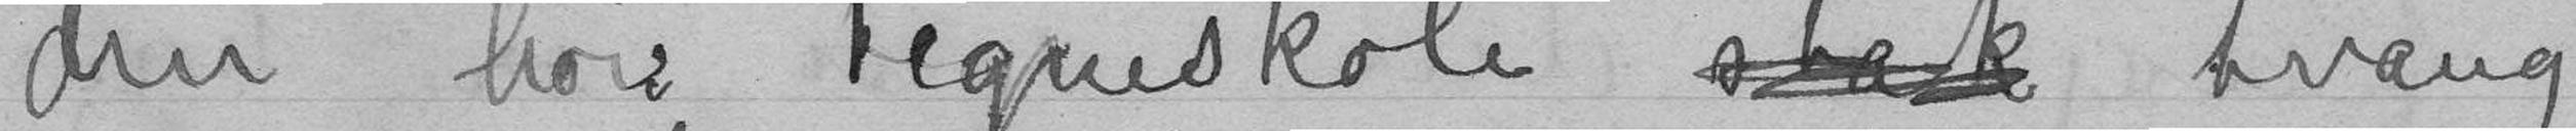den høie tegneskole stak tvang
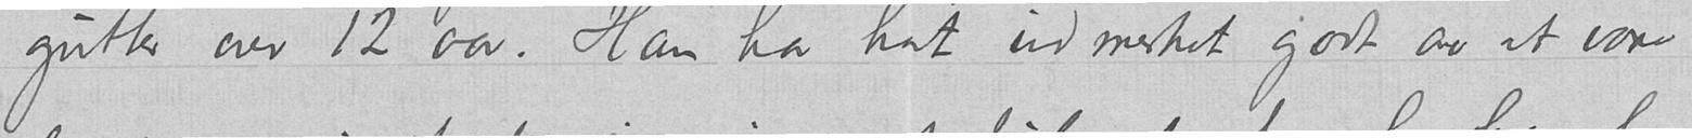gutter over 12 aar. Han har hat udmerket godt av at være
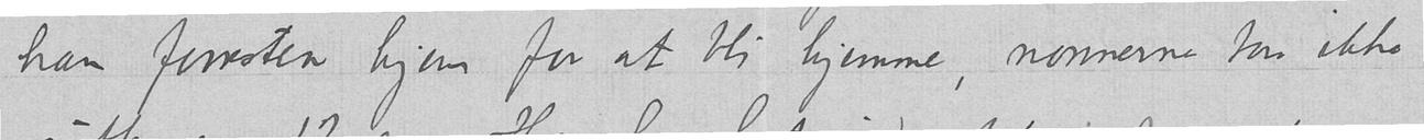han forresten hjem for at bli hjemme, nonnerne tar ikke
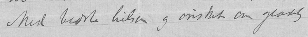Med bedste hilsen og ønsket om glædelig
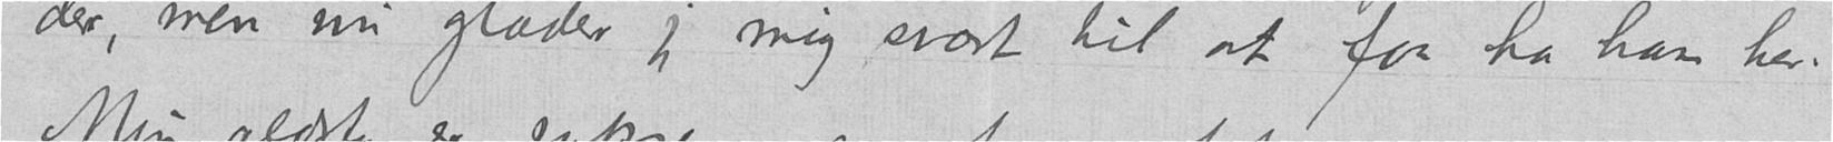der, men nu glæder jeg mig svært til at faa ha ham her.
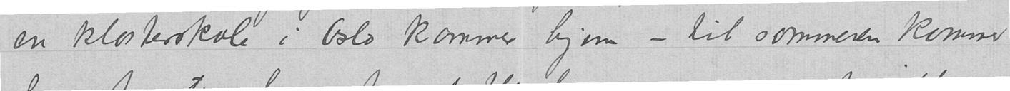en klosterskole i Oslo kommer hjem - til sommeren kommer
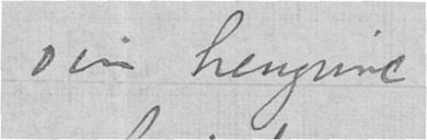Din hengivne
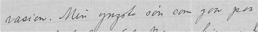vasion. Min yngste søn som gaar paa
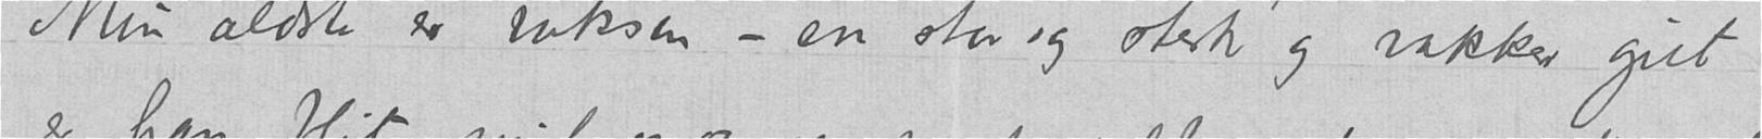Min ældste er voksen - en stor og sterk og vakker gut
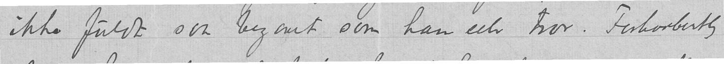ikke fuldt saa begavet som han selv tror. Forhaabentlig
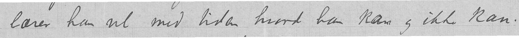lærer han vel med tiden hvad han kan og ikke kan.
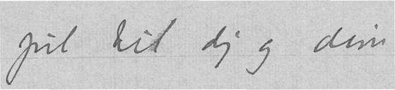jul til dig og dine
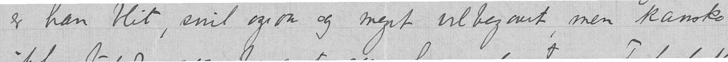er han blit, snil ogsaa og meget velbegavet, men kanske
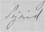Sigrid
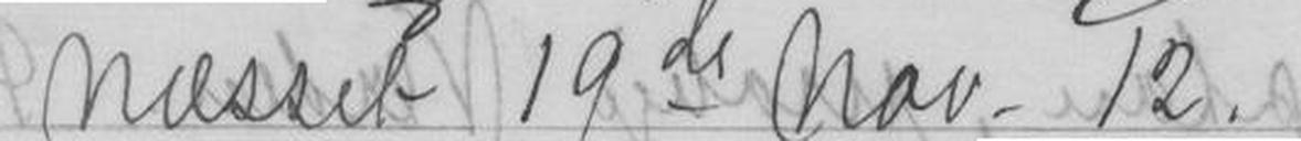Næsset 19de Nov. 12
